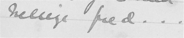hellige fred...
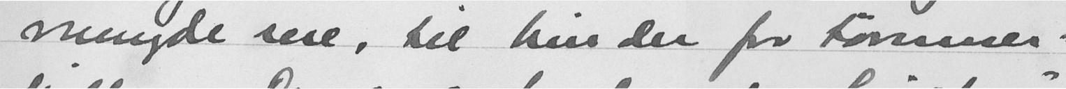mengde sne, til hen der for tømmer-
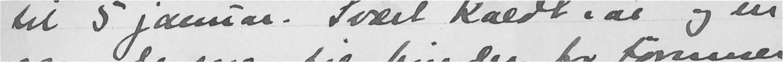til 3 januar. Svært koldt iår og en
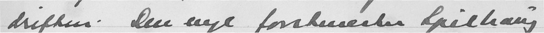driften. Den nye forstenester Spilhaug
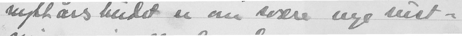nyttårsbudet er om svære nye rust-
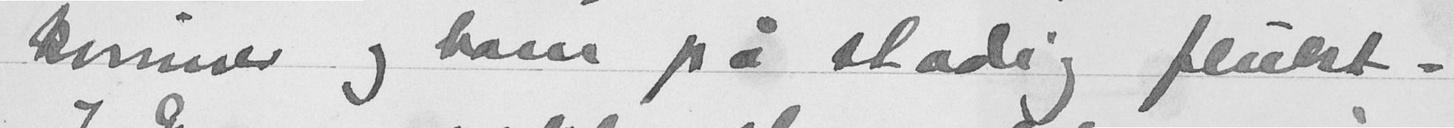kvinner og barn på stadig flukt.
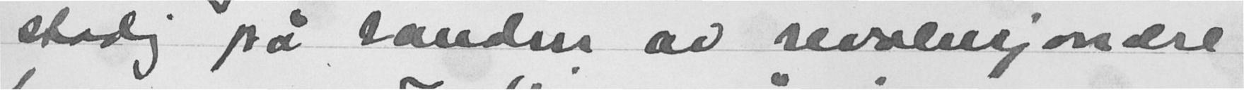stadig på randen av revolusjonære
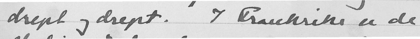drept og drept. I Frankrike er de
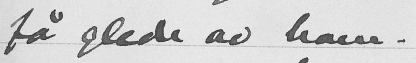få glede av ham.
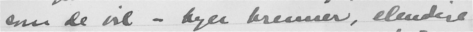som de vil - byer brenner, elendige
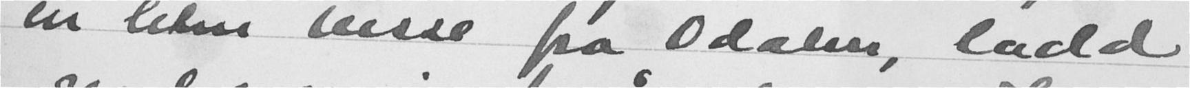en liten reisse få Odalen, ladd
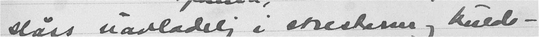slåss uavladelig i snestorm og kulde -
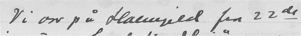Vi var på Holmgild fra 22de
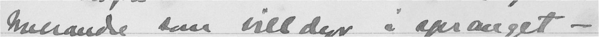hverandre som villdyr i spranget -
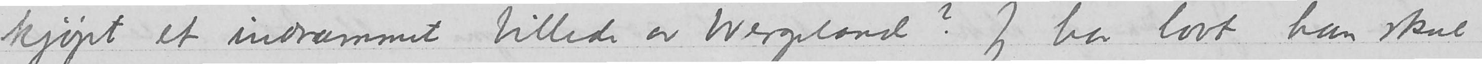kjøpt et indrammet billede av Wergeland? Jeg har lovt han skal
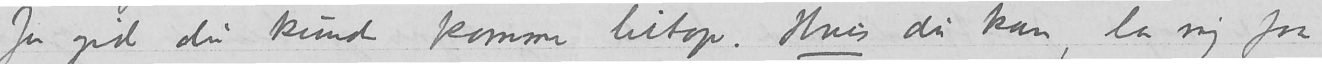Ja gid du kunde komme hitop. Hvis du kan, la mig faa
